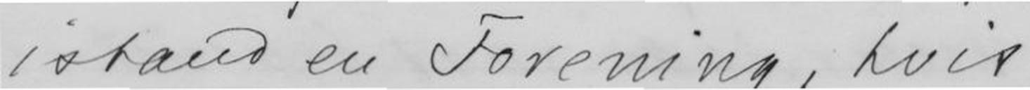istand en Forening, hvis
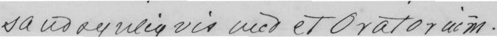sandsynligvis med et Oratorium.
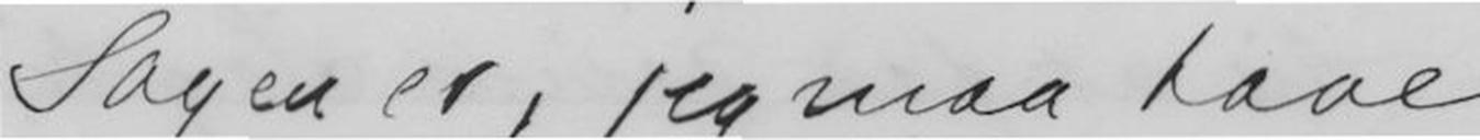Sagen er, jeg maa have
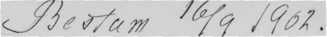Bestum 16/9 1902
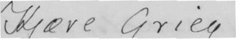Kjære Grieg
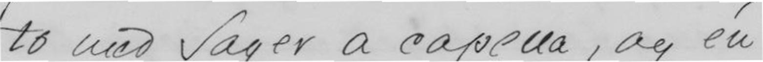to med Sager a capella, og en
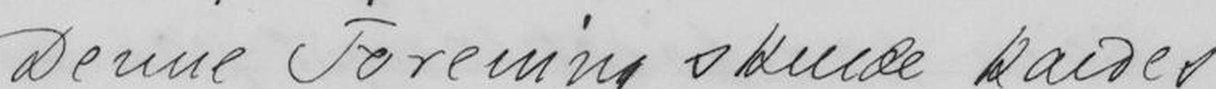Denne Forening skulde kaldes
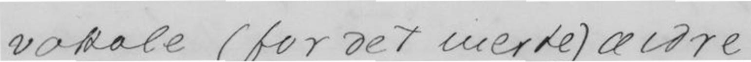vokale (for det merke) ældre
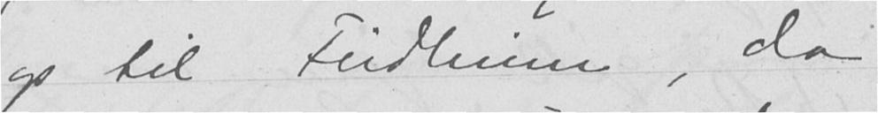op til Fridheim, da
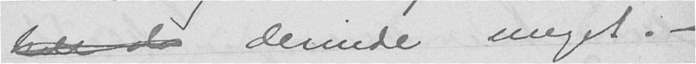her da derinde meget. -
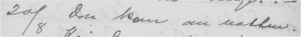20/8 Don kom om natten.
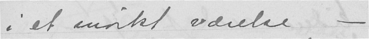i et mørkt værelse -
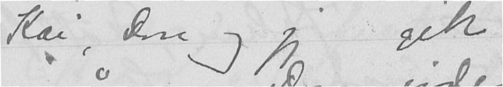Kai, Don og jeg gik
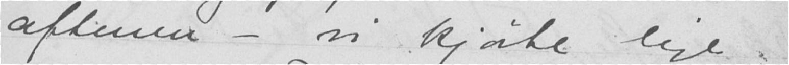aftenen - vi kjørte lige
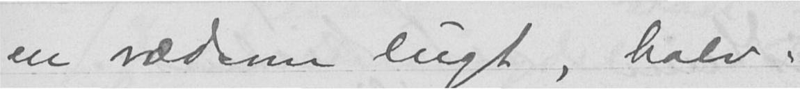en rædsom lugt, halv-
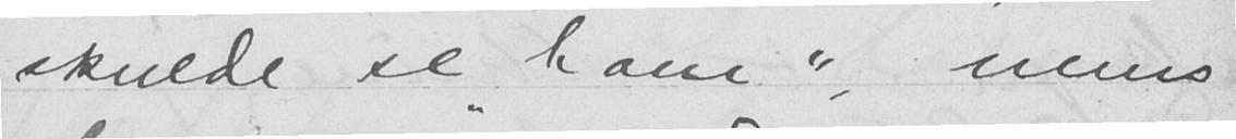skulde se "ham", mens
